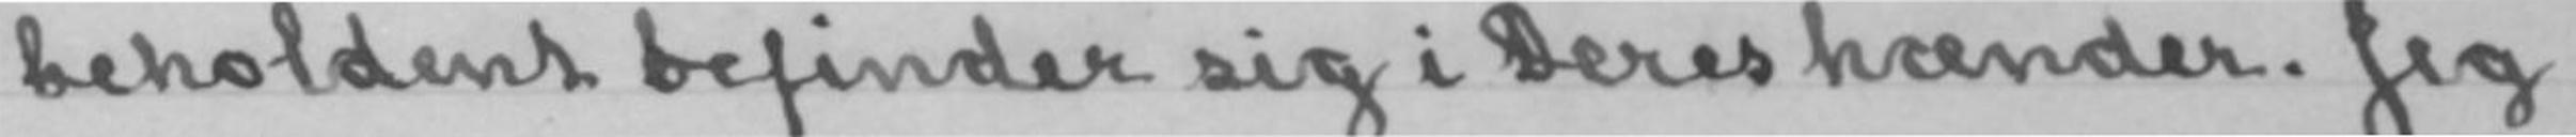beholdent befinder sig i Deres hænder. Jeg
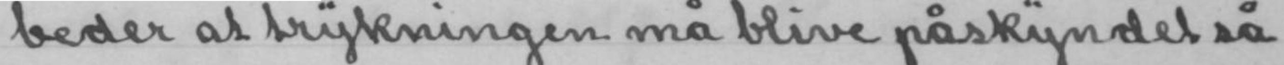beder at trykningen må blive påskyndet så
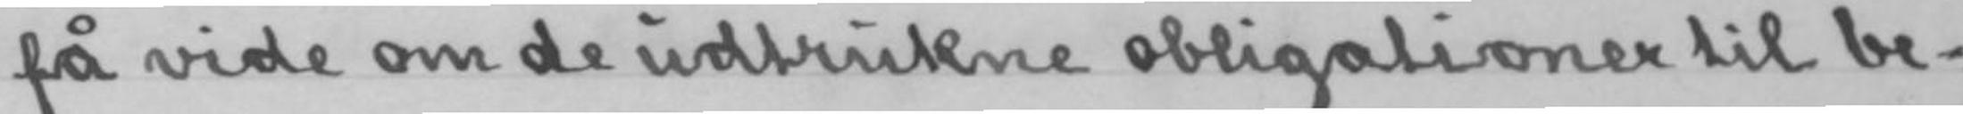få vide om de udtrukne obligationer til be-
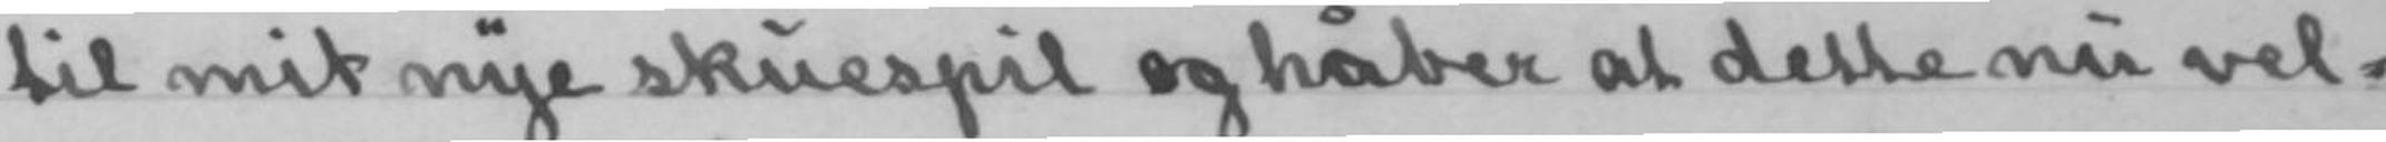til mit nye skuespil og håber at dette nu vel-
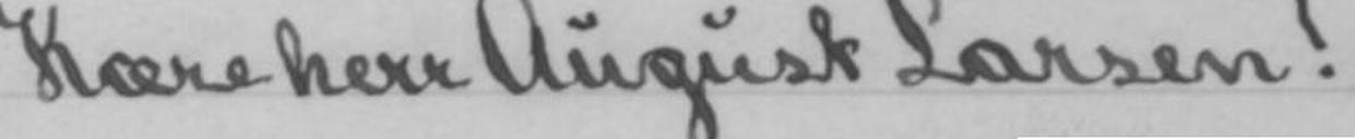Kære herr August Larsen!
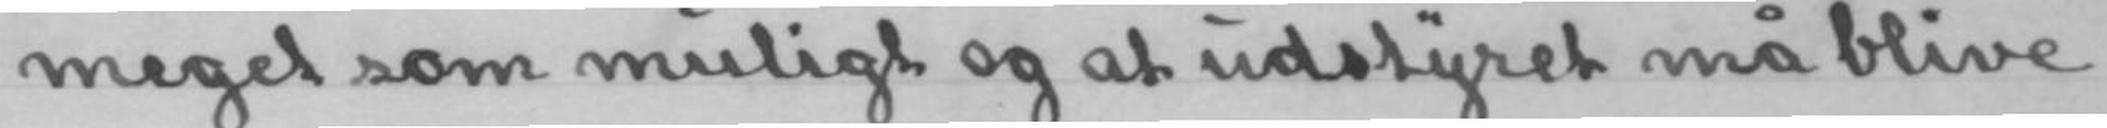meget som muligt og at udstyret må blive
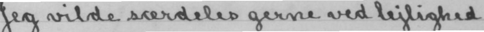Jeg vilde særdeles gerne ved lejlighed
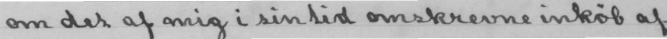om det af mig i sin tid omskrevne inkøb af
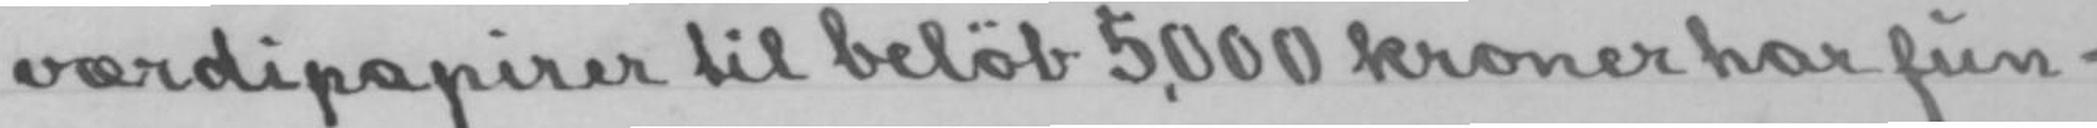værdipapirer til beløb 5,000 kroner har fun-
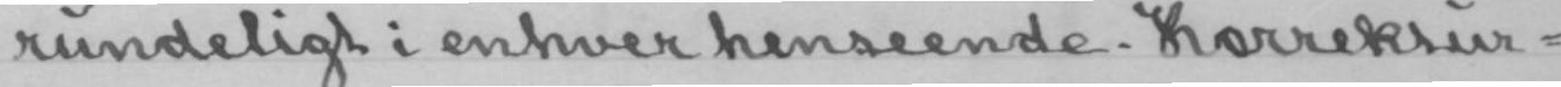rundeligt i enhver henseende. Korrektur-
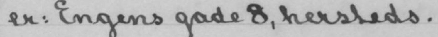er: Engens gade 8, hersteds.
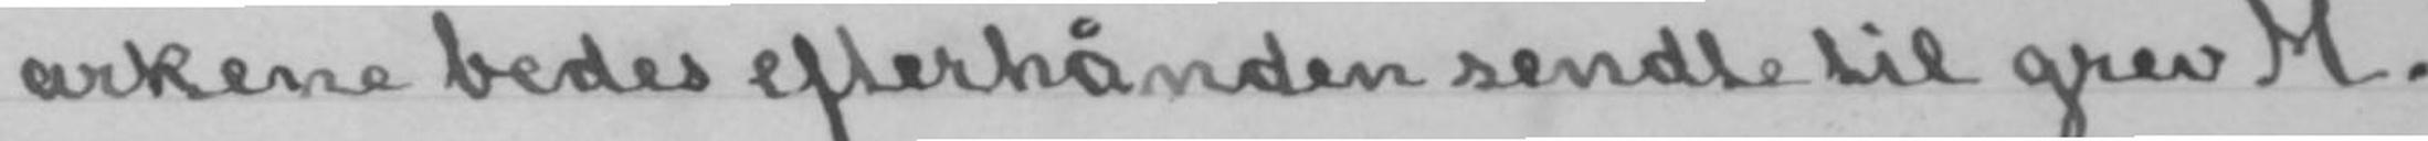arkene bedes efterhånden sendte til grev M.
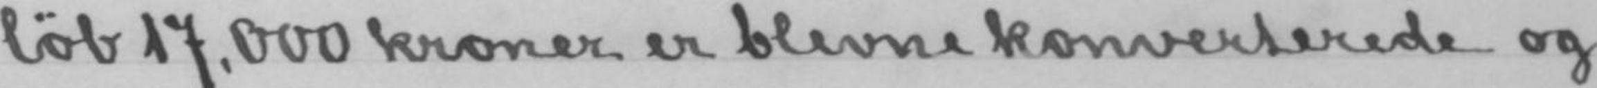løb 17,000 kroner er blevne konverterede og
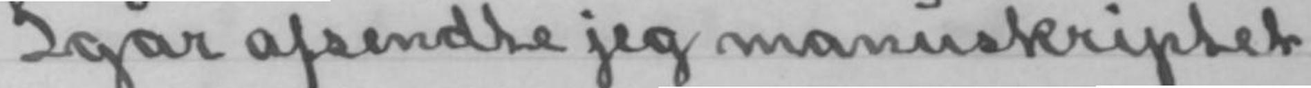Igår afsendte jeg manuskriptet
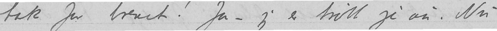tak for brevet! Ja – jeg er trøtt jeg au. Nu
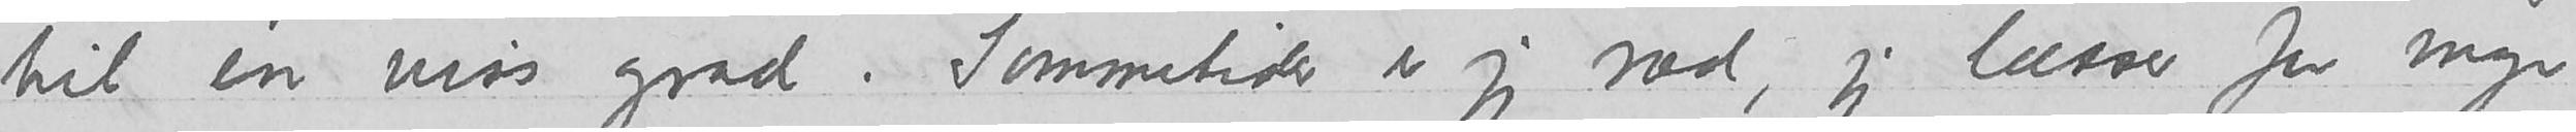til en viss grad. Sommetider er jeg ræd, jeg læsser for mye
